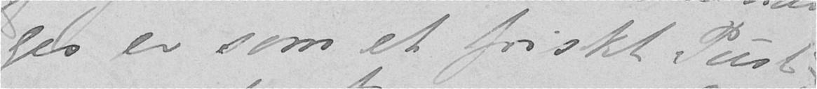ges er som et friskt Pust,
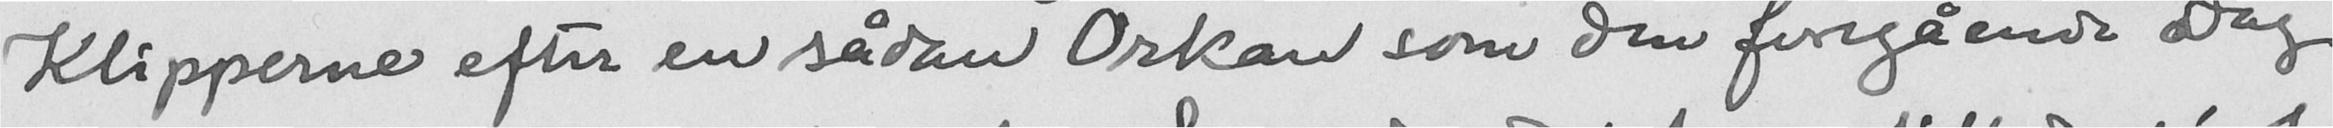Klipperne efter en sådan Orkan som den foregående Dag
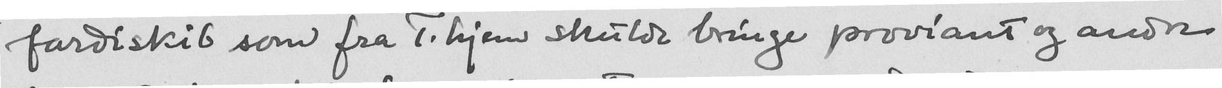fardiskib som fra T.hjem skulde bringe proviant og andre
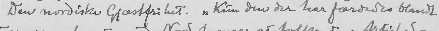Den nordiske Gjæstfrihet. "Kun den der har færdedes blandt
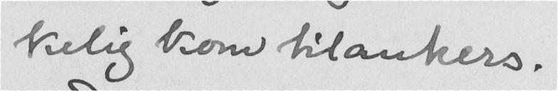kelig kom tilankers.
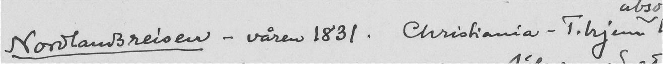Nordlandsreisen - våren 1831. Christiania - T.hjem
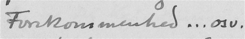Forekommenhed.. osv.
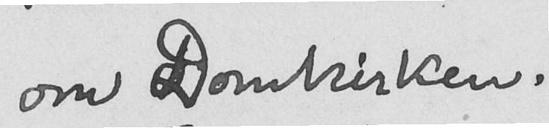om Domkirken.
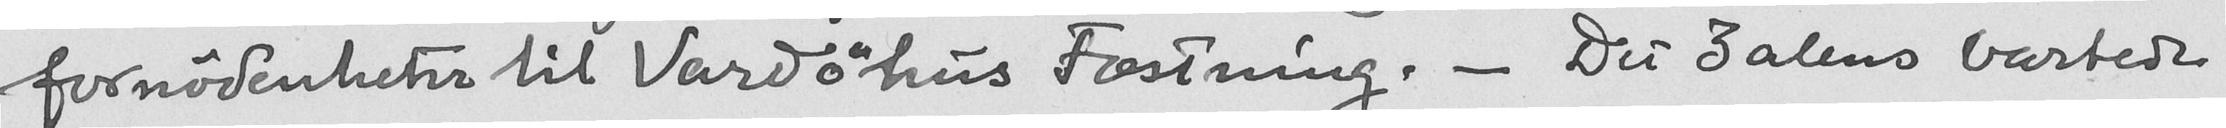fornødenheter til Vardøhus Fæstning. - Det Zalens bartede
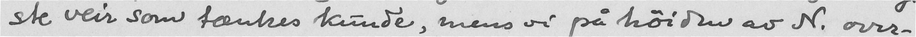ste veir som tænkes kunde, mens vi på høiden av N. over-
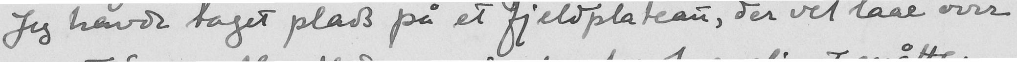Jeg havde taget plads på et Fjeldplateau, der vel laae over
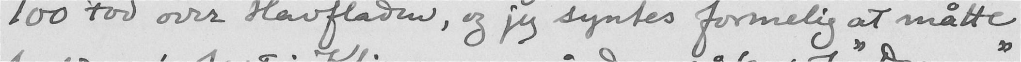100 Fod over Havfladen, og jeg syntes formelig at måtte
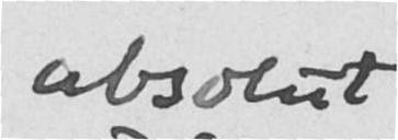absolut
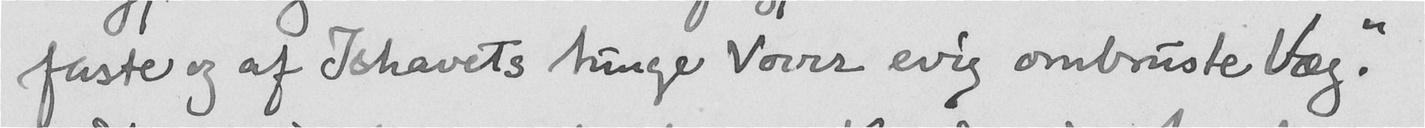faste og af Ishavets tunge Vover evig ombruste Væg."
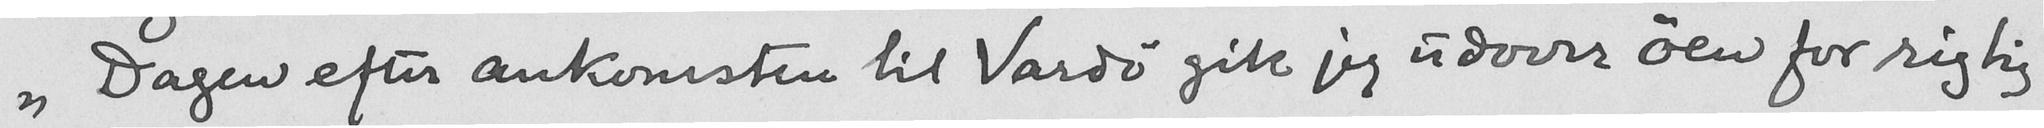"Dagen efter ankomsten til Vardø gik jeg udover øen for rigtig
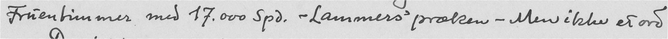Fruentimmer med 17.000 spd. - Lammers' præken - Men ikke et ord
